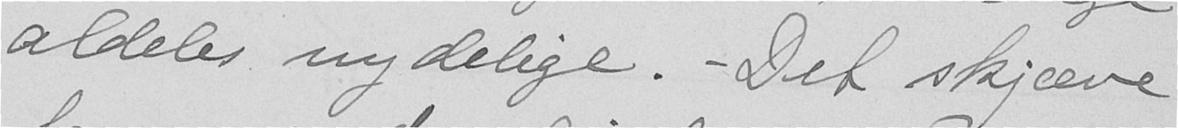aldeles nydelige. - Det skjæve
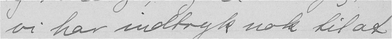vi har indtryk nok til at
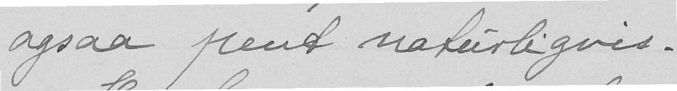ogsaa pent naturligvis.
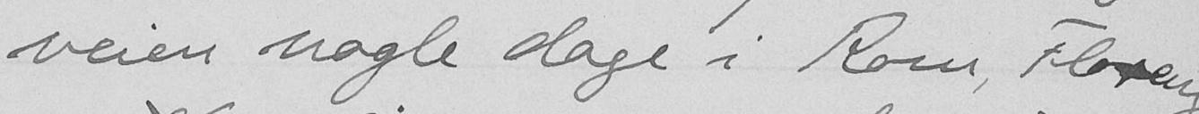veien nogle dage i Rom, Florens
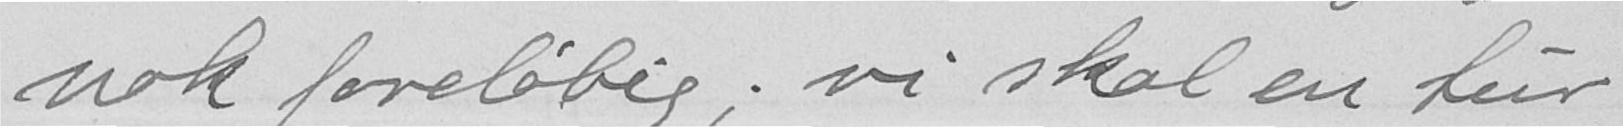nok foreløbig, vi skal en tur
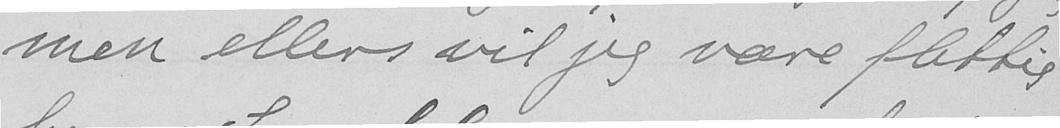men ellers vil jeg være flittig
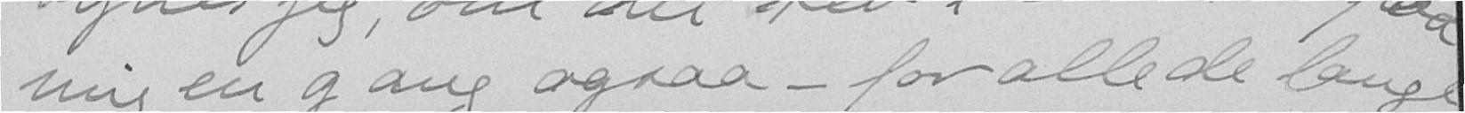mig en gang ogsaa- for alle de lange
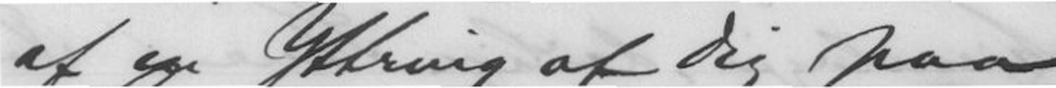af en Yttring af dig paa
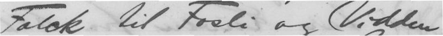Falck til Fosli og Vidden
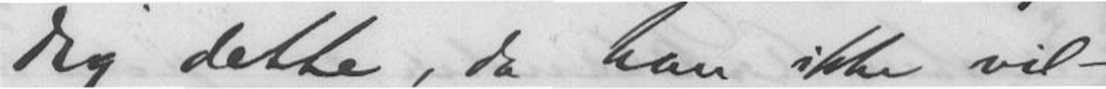dig dette, da han ikke vil-
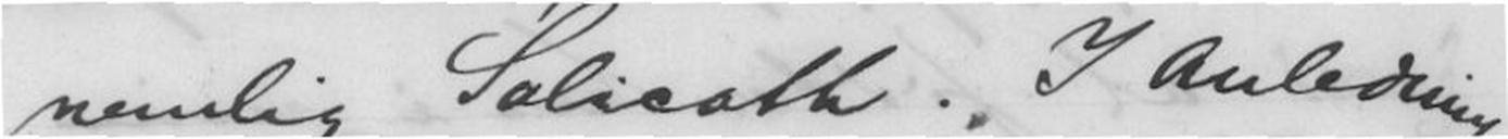nemlig Salicath. I Anledning
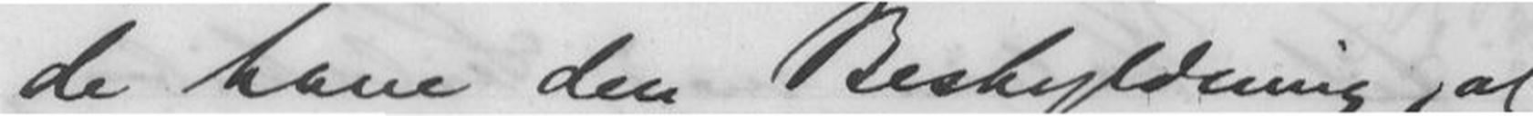de have den Beskyldning, at
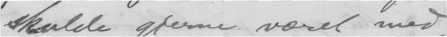skulde gjerne været med,
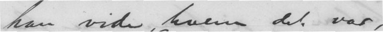han vide, hvem det var,
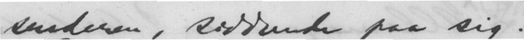senderen, siddende paa sig.
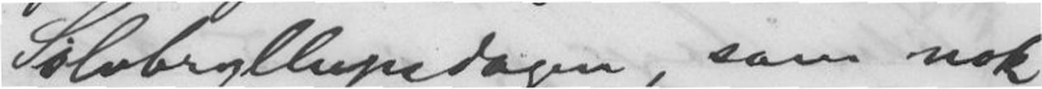Sølvbryllupsdagen, som nok
